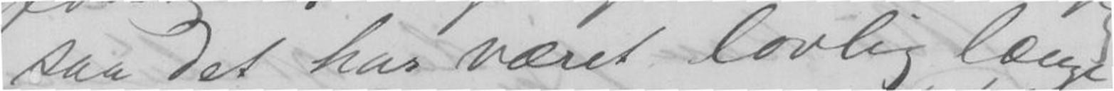saa det har været lovlig længe
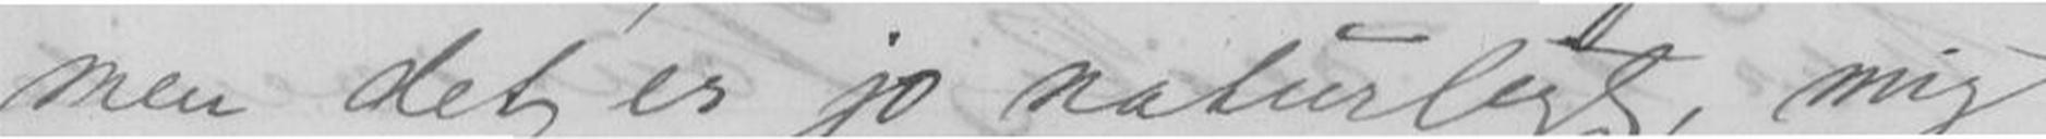men det er jo naturligts, mig
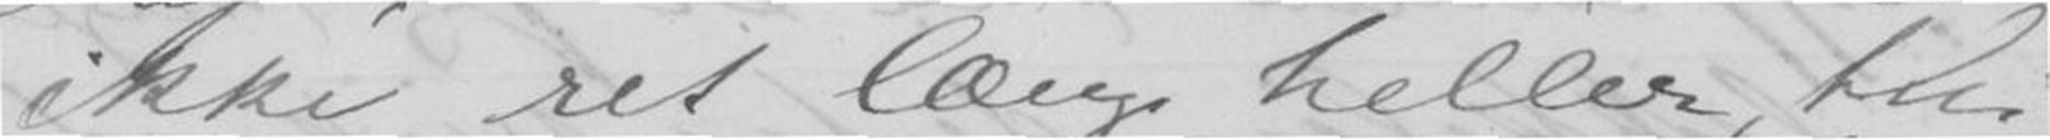ikke ret længe heller, thi
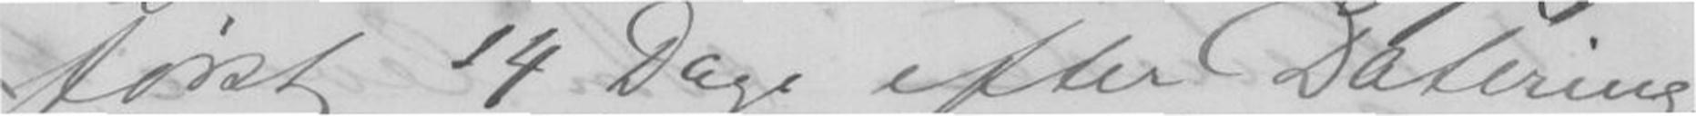først 14 Dage efter Datering
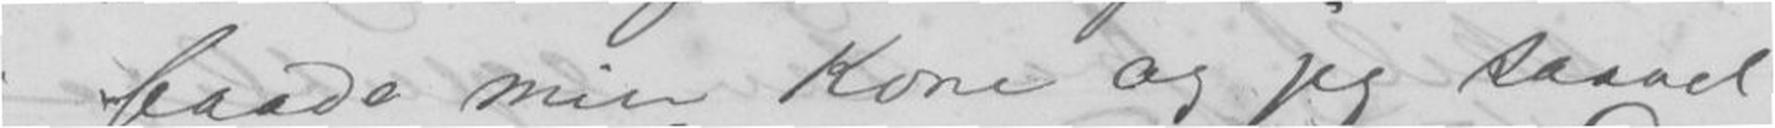baade min Kone og jeg saavel
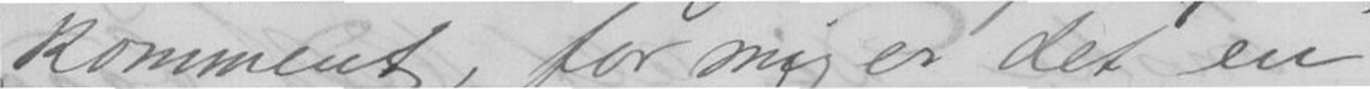kommet, for mig er det en
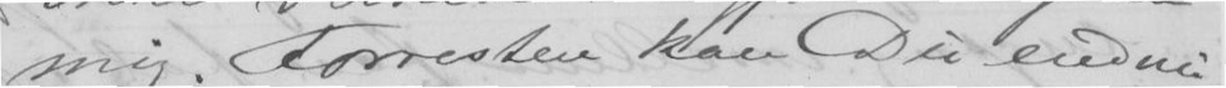mig. Forresten kan Du endnu
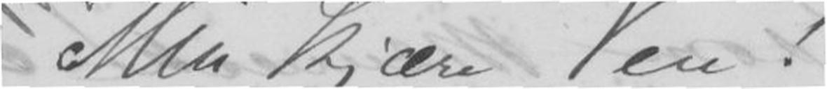Min Kjære Ven!
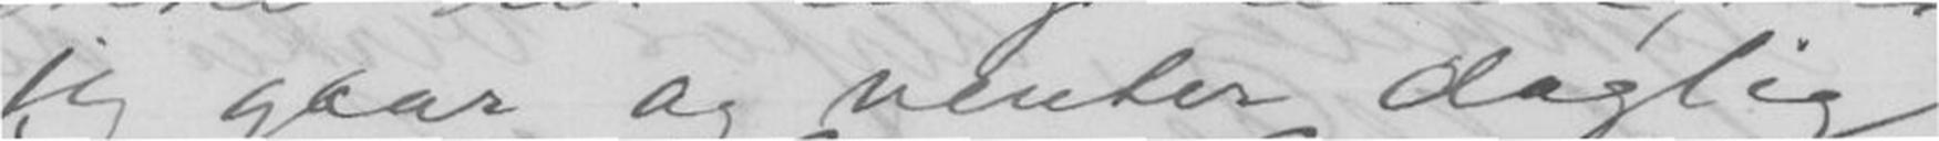jeg gaar og venter daglig
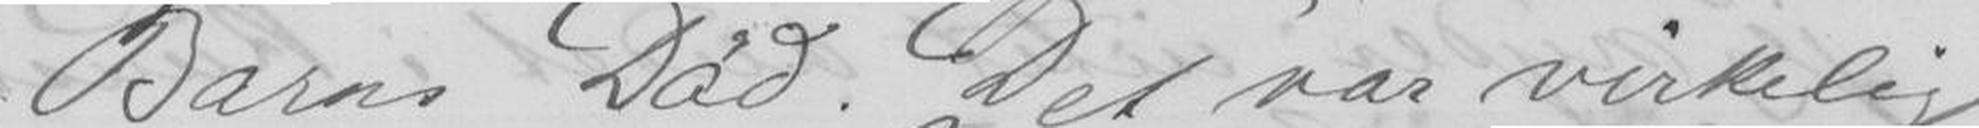Barns Død. Det var virkelig
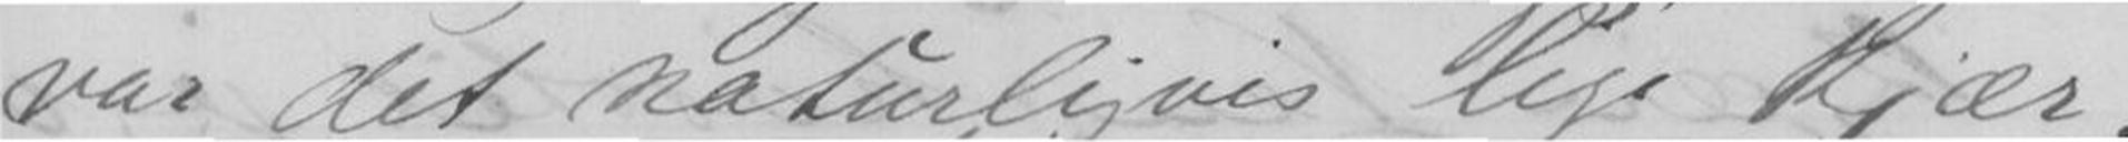var det naturligvis lige kjær-
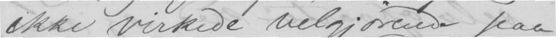ikke virkede velgjørende paa
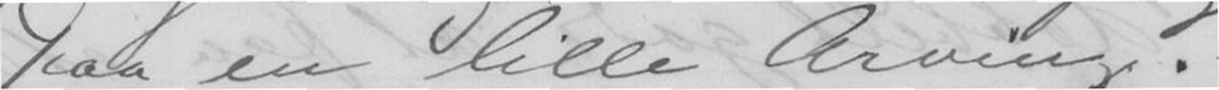paa en lille Arving.-
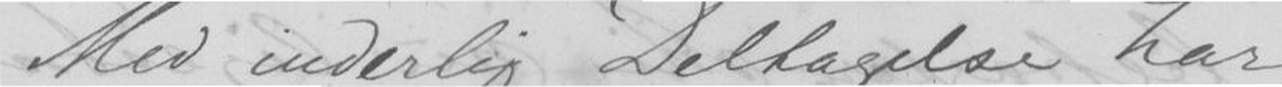Med inderlig Deltagelse har
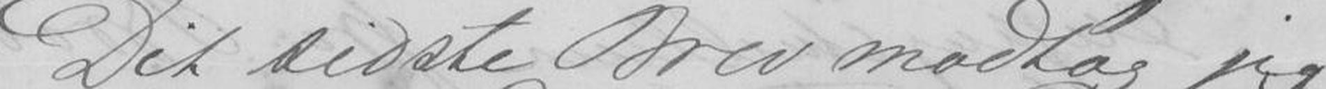Det sidste Brev modtog jeg
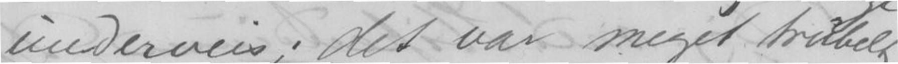underveis, det var meget trubelt
